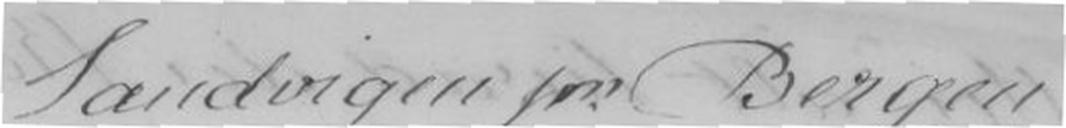Sandvigen pr. Bergen
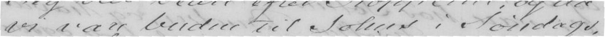vi vare bunden til Johns i Søndags
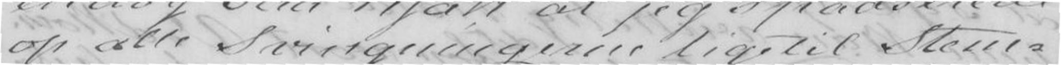op alle svigningerne ligetil Stem-
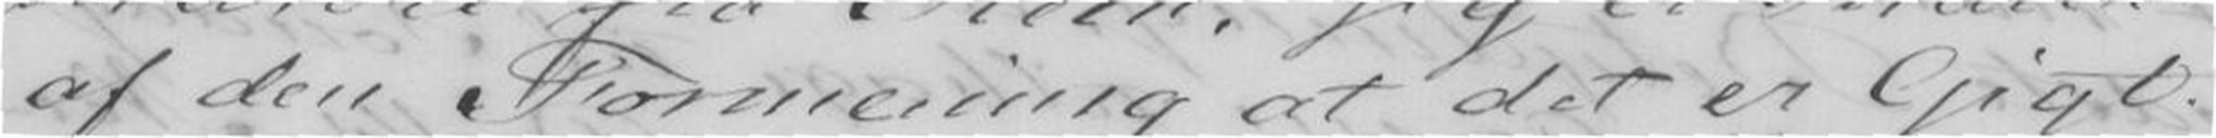af den Formening at det er Gigt. -
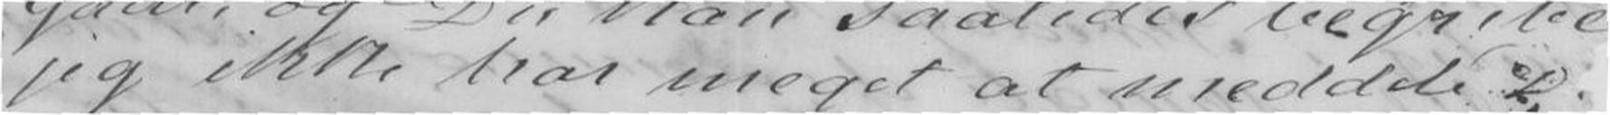jeg ikke har meget at meddele Di
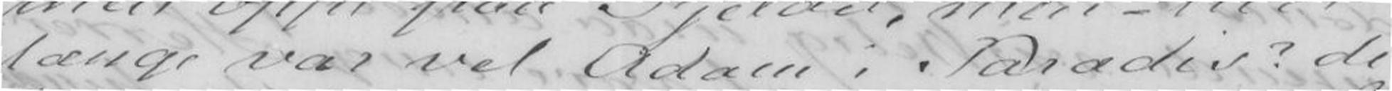læmge var vel Adam i Paradis? de
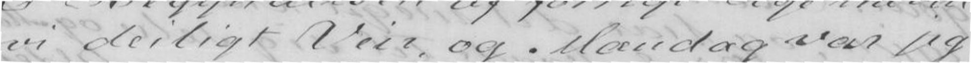vi deiligt Veir, og Mandag var jeg
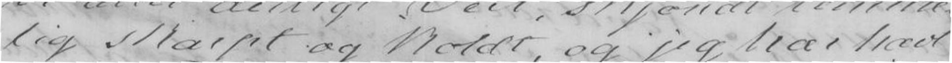lig skarpt og koldt, og jeg har havt
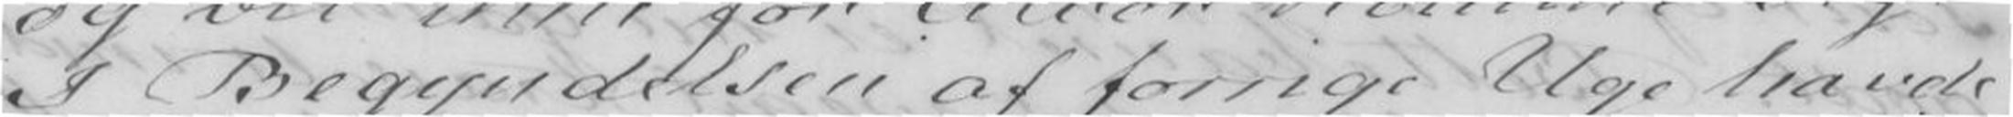I Begyndelsen af forrige Uge havde
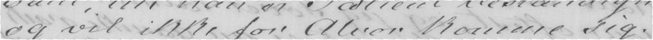og vil ikke for Alvor komme sig.
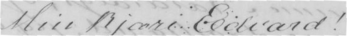Min kjære Edvard!
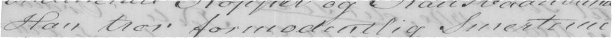Han tror formodentlig Smerterne
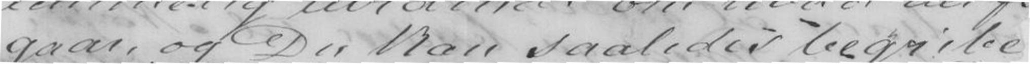gaar, og Du kan saaledes begribe
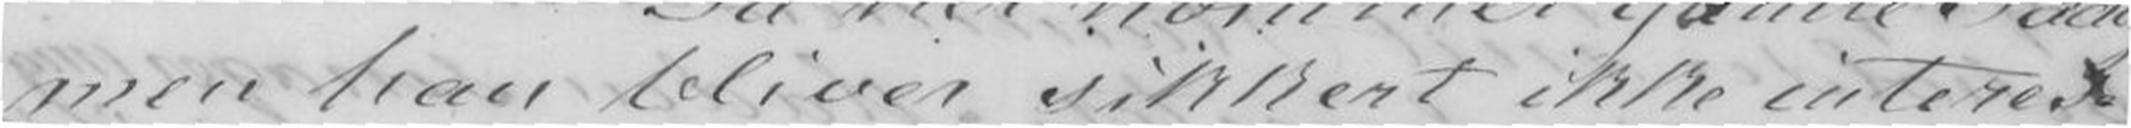men han bliver sikkert ikke interes-
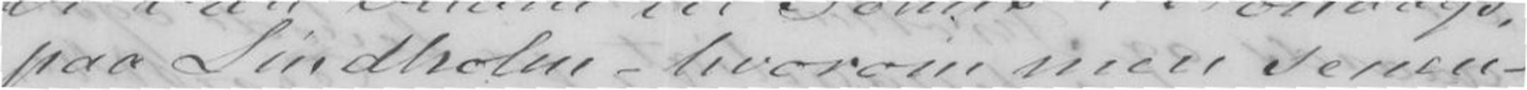paa Lindholm - hvorom mere senere -
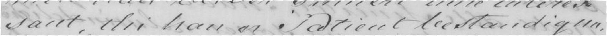sant, thi han er Patient bestandig nu,
